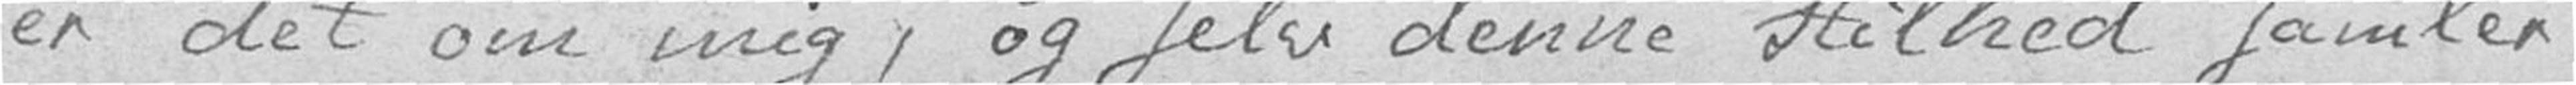er det om mig, og selv denne Stilhed samler
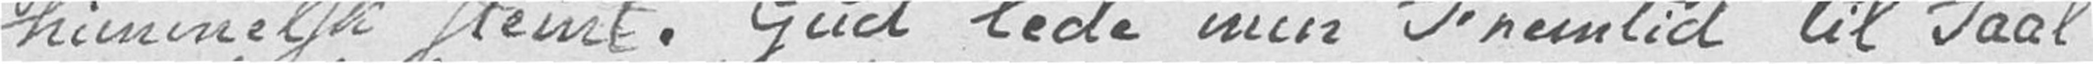himmelsk stemt. Gud lede min Fremtid til Taal
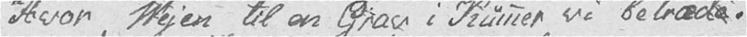Hvor Vejen til en Grav i Kummer vi betræde.
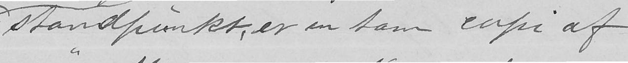standpunkt, er en tam copi af
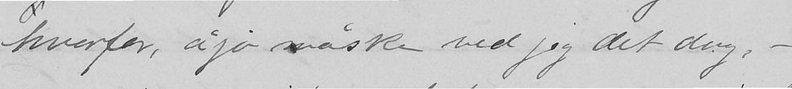hvorfor, åjo måske ved jeg det dog. -
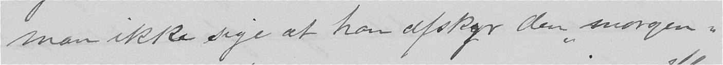man ikke sige at han afskyr den "morgen-
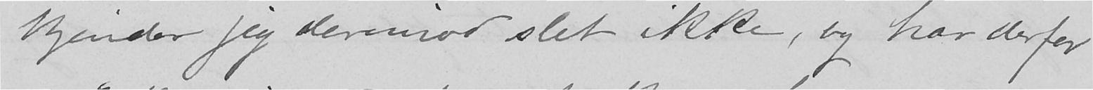kjender jeg derimod slet ikke, og har derfor
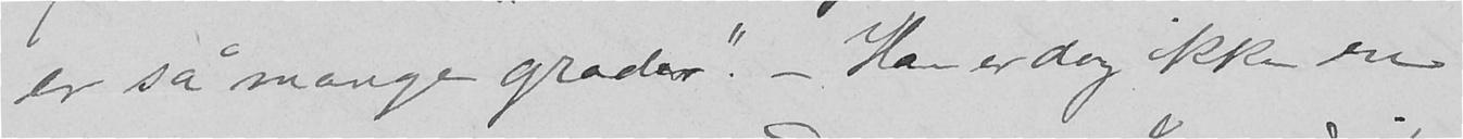er sa mange grader". - Han er dog ikke en
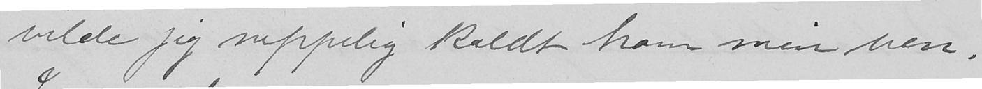vilde jeg neppelig kaldt ham min ven.
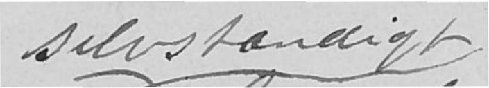selvstendigt
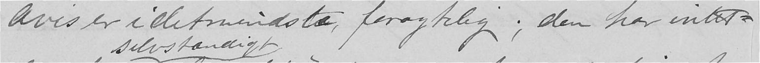avis er idetmindste, foragtelig; den har intet-
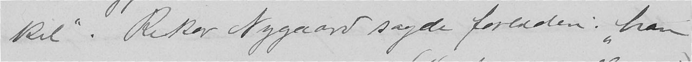kel". Rektor Nygaard sagde forleden: "han
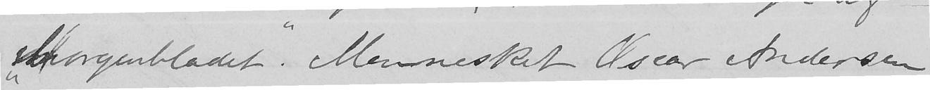"Morgenbladet". Mennesket Oscar Andersen
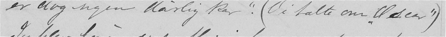er dog ingen dårlig kar". (Vi talte om "Oscar")
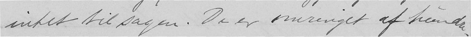intet til sagen. De er omringet af hundre-
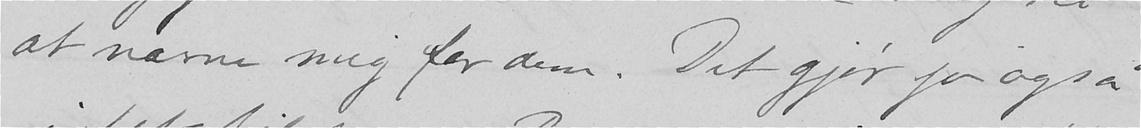at nævne mig for dem. Det gjør jo også
